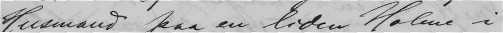Husmand paa en liden Holme i
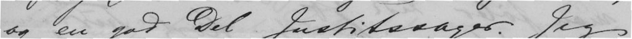og en god Del Justitssager. Jeg
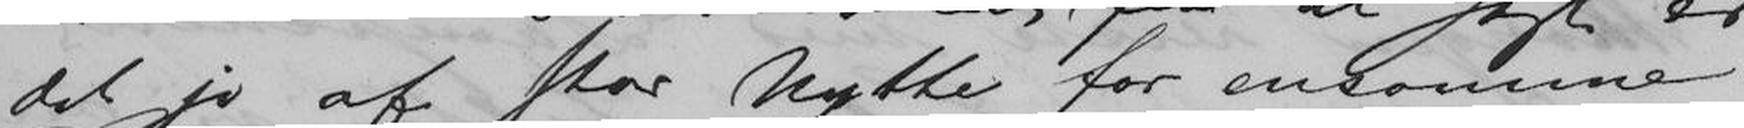det jo af stor Nytte for ensomme
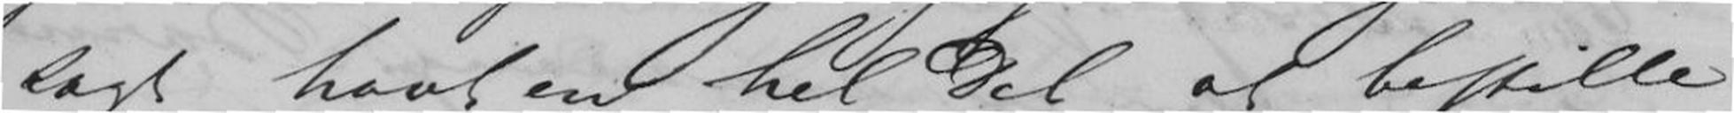sagt havt en hel Del at bestille
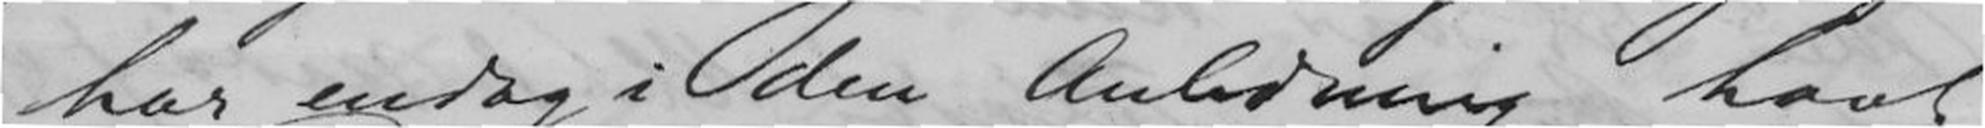har endog i den Andledning havt
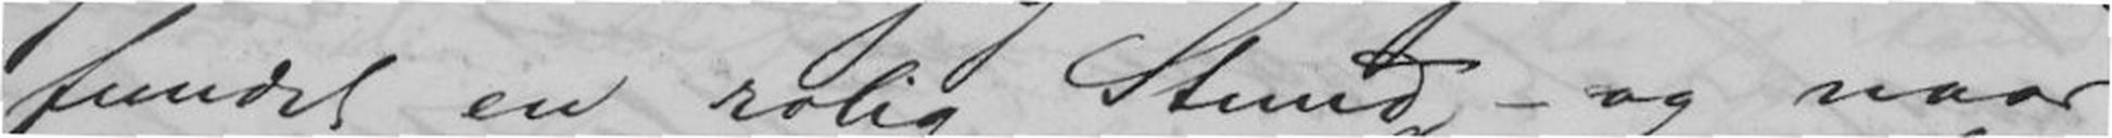fundet en rolig Stund - og naar
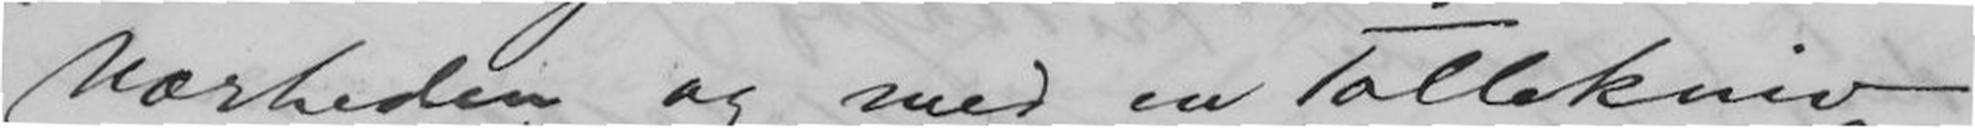Nærheden og med en Tollekniv
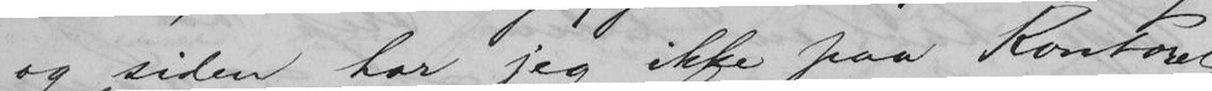og siden har jeg ikke paa Kontoret
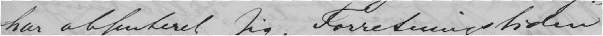har absenteret sig, Forretningstiden
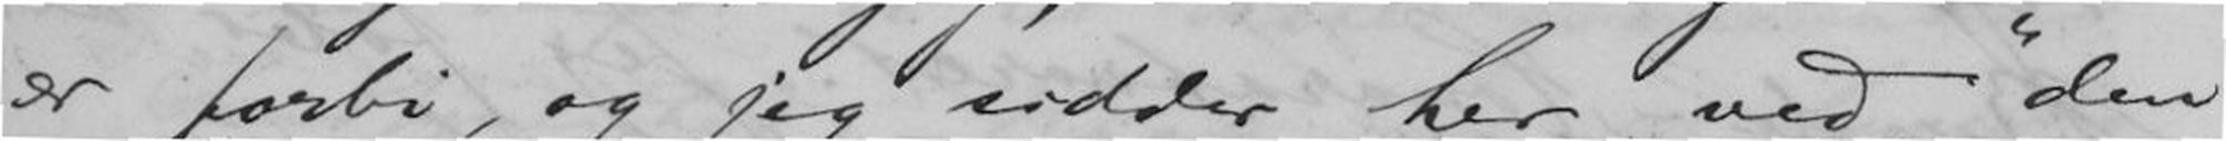er forbi, og jeg sidder her ved "den
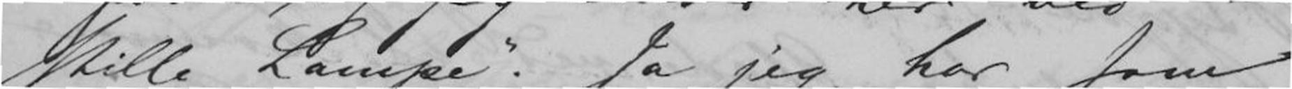stille Lampe". Ja jeg har som
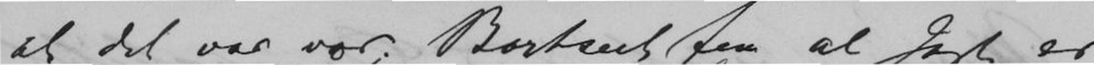at det var vor. Bortseet fra al Jagt er
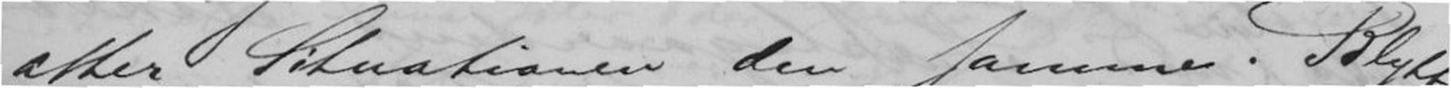atter Situationen den samme. Blytt
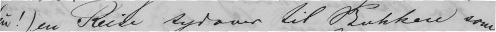en Reise sydover til Bukken som
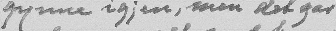gynne igjen, men det går
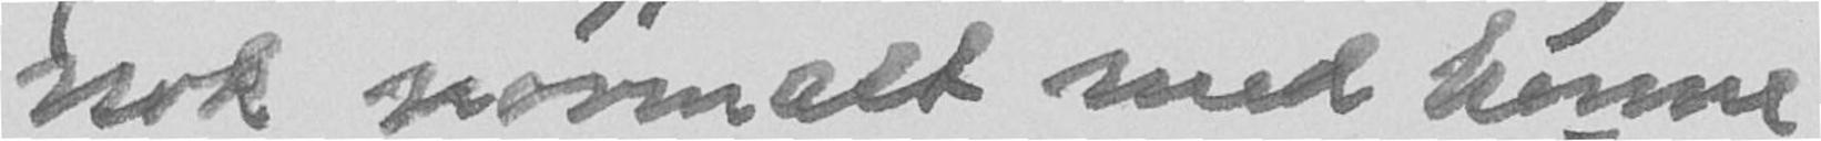nok normalt med henne
